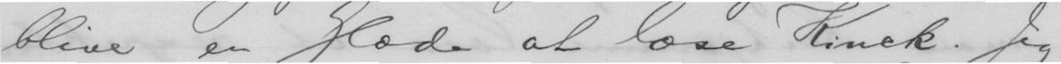blive en Glæde at læse Kinck. Jeg
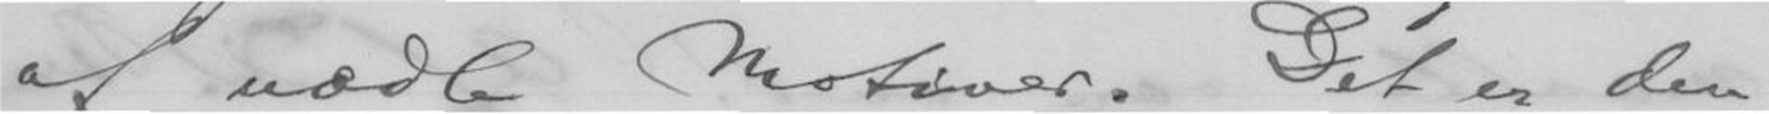af uædle Motiver. Det er den-
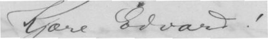Kjære Edvard!
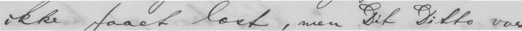ikke faaet læst, men Dit Ditto var
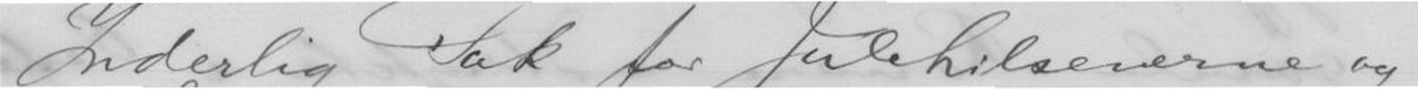Inderlig Tak for Julehilsenerne og
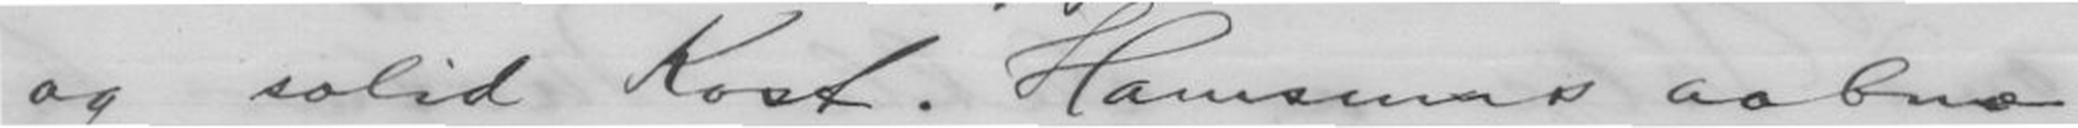og solid Kost. Hamsuns aabne
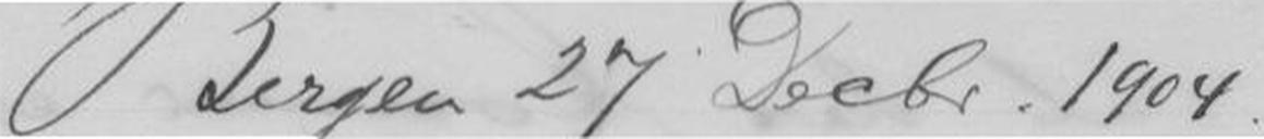Bergen 27 Decbr. 1904.
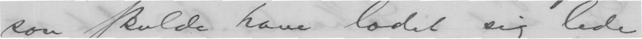son skulde have ladet sig lede
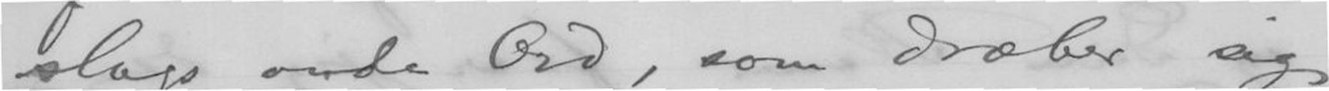slags onde Ord, som dræber sig
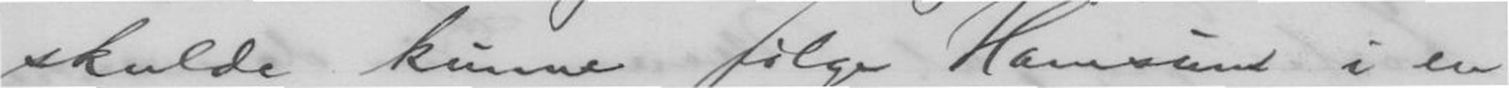skulde kunne følge Hamsun i en
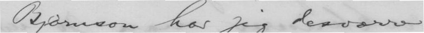Bjørnson har jeg desværre
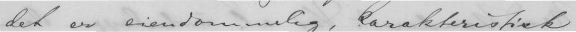det er eiendommelig, karakteristisk
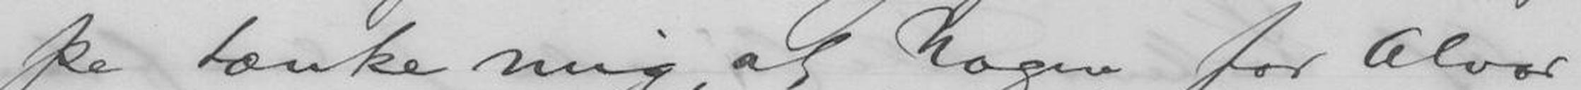pe tænke mig, at Nogen for Alvor
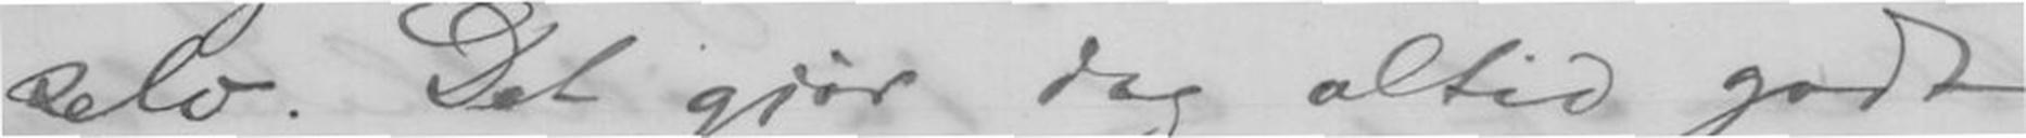selv. Det gjør dog altid godt
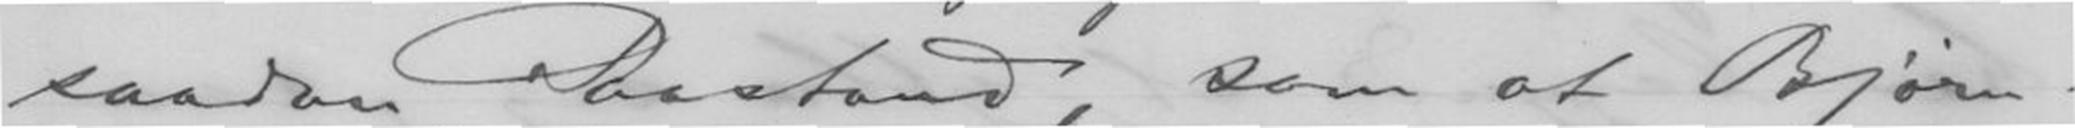saadan Paastand, som at Bjørn-
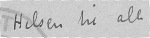Hilsen til alle
